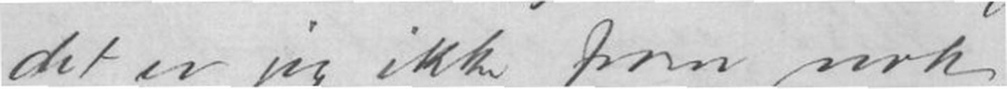det er jeg ikke fram nok
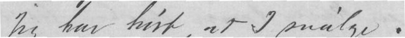jeg har hørt, at I
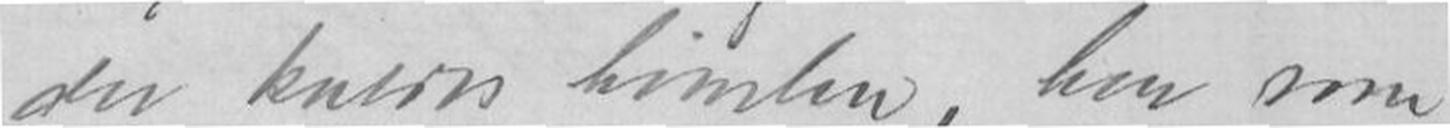der kaldes himlen, kom som
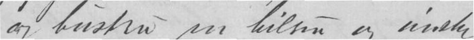og hustru en hilsen og ønsker
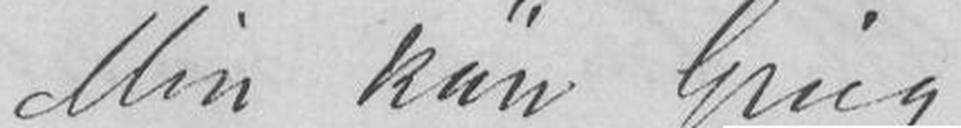Min kære Grieg
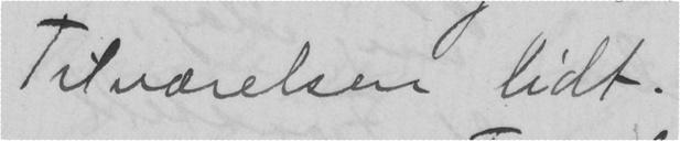Tilværelsen lidt.
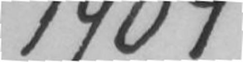1904
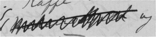og
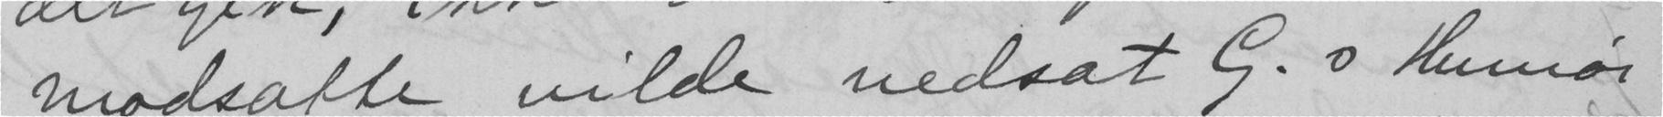modsatte vilde nedsat G.s Humør.
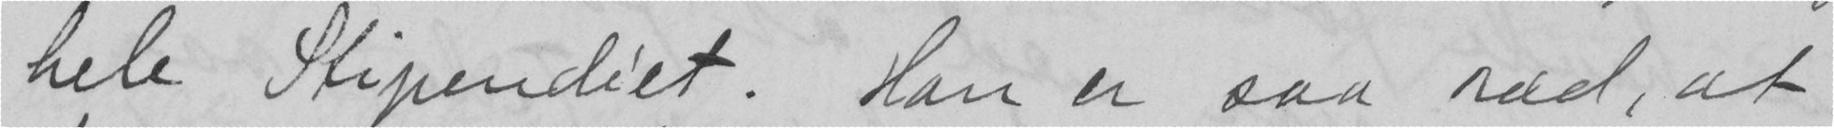hele Stipendiet. Han er saa ræd, at
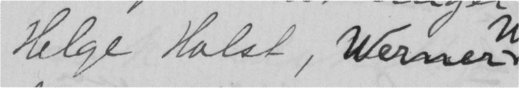Helge Holst, Werner
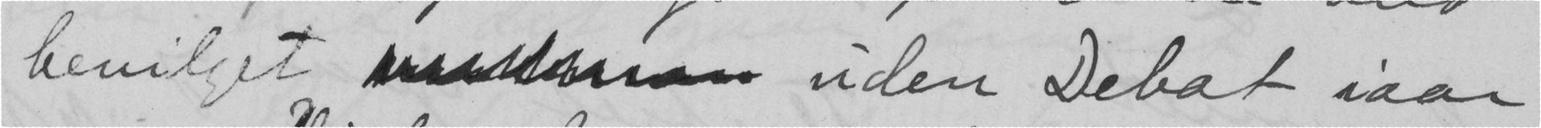bevilget  uden Debat iaar
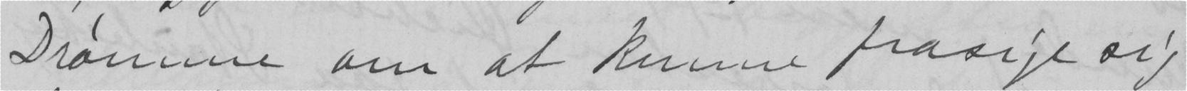Drømme om at kunne frasige sig
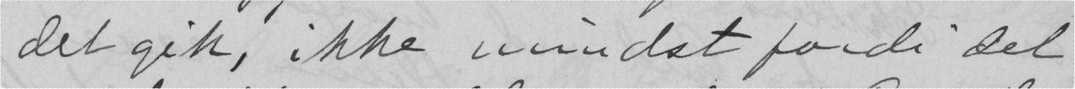det gik, ikke mindst fordi det
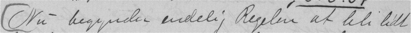Nu begynder endelig Regelen at bli lidt
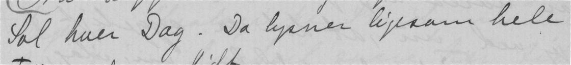Sol hver Dag. Da lysner ligesom hele
